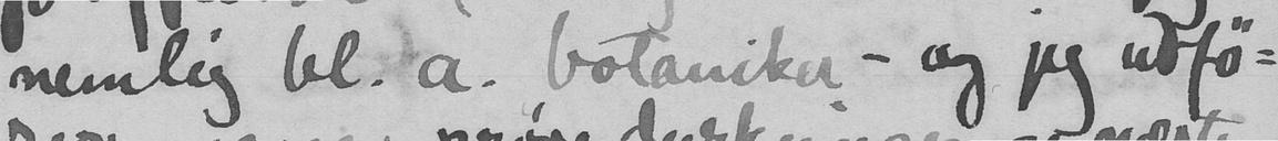nemlig bl. a. botaniker - og jeg udfø-
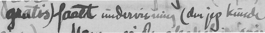gratis) faaet undervisning (den jeg kunde
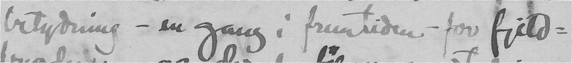betydning - en gang i fremtiden - for fjeld-
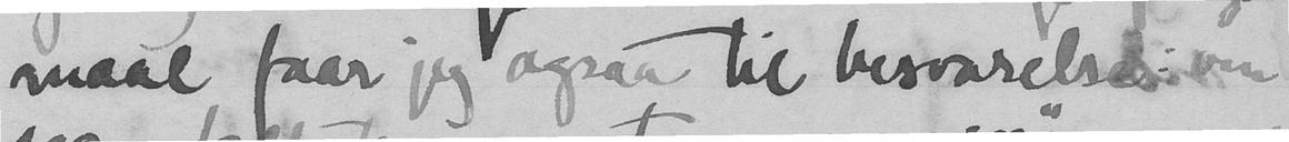maal faar jeg ogsaa til besvarelse: om
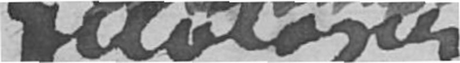filologer
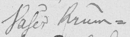Vaser Krum-
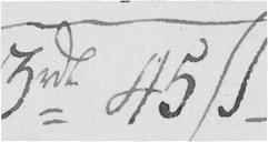3 rde 45 s
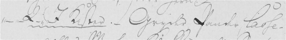"- "-2-" I Kister. - Gryder, Pander, Casse-
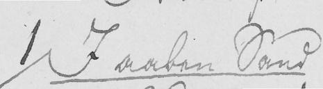1 I aaben Sand
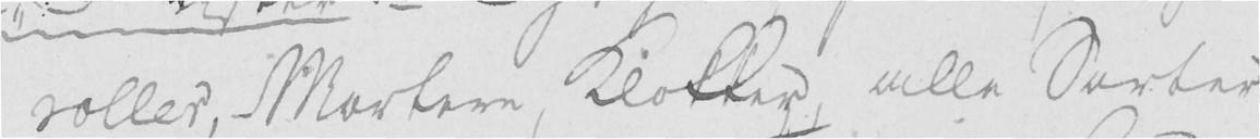roller, Mortere, Klokker, alle Sorter
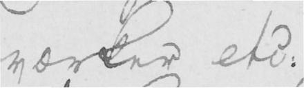værker etc.
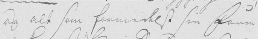og alt som formedelst sin Form
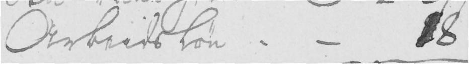Arbeidsløn - - 18
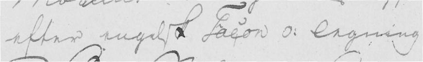efter engelsk Facon o. legning
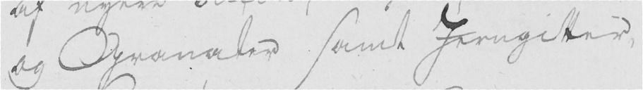og Granater samt Jerngitter,
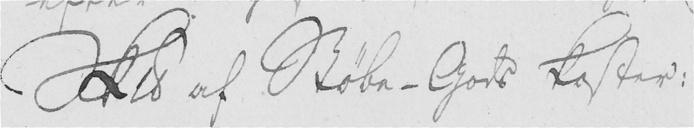Skpd af Støbe-Gods koster:
